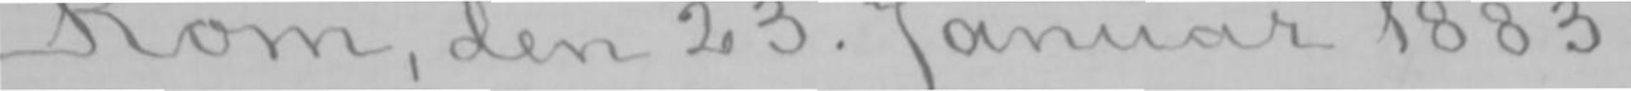Rom, den 23. Januar 1883.
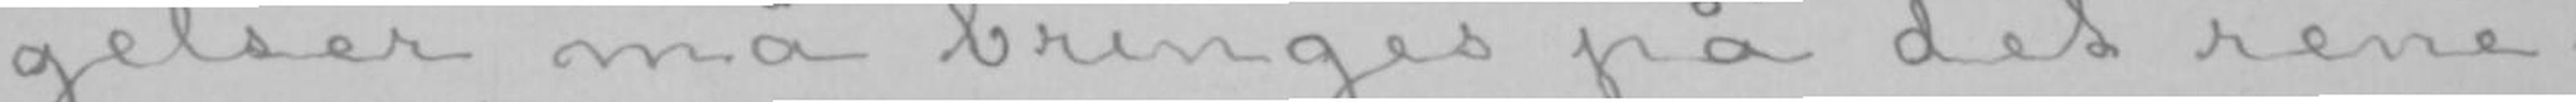gelser må bringes på det rene;
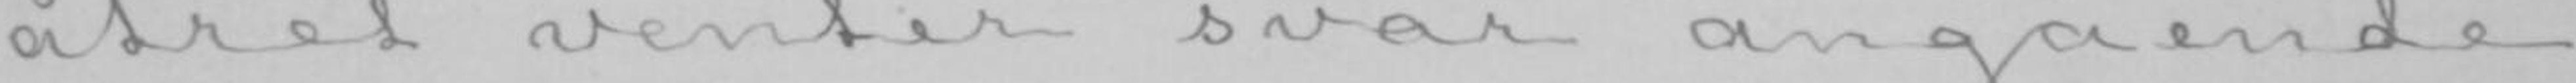atret venter svar angående
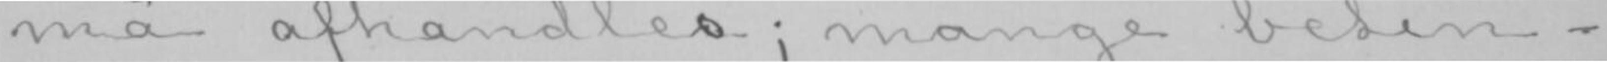må afhandles; mange betin-
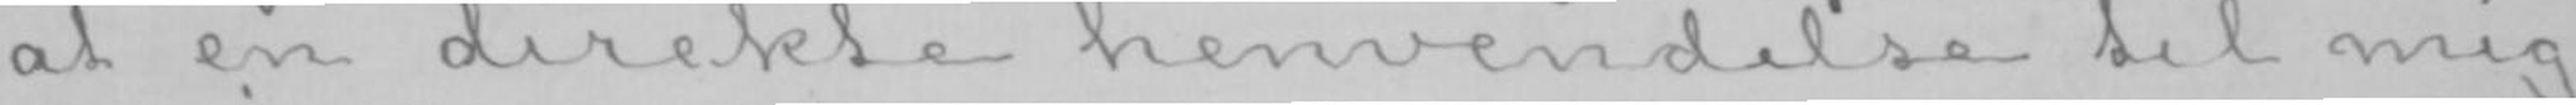at en direkte henvendelse til mig
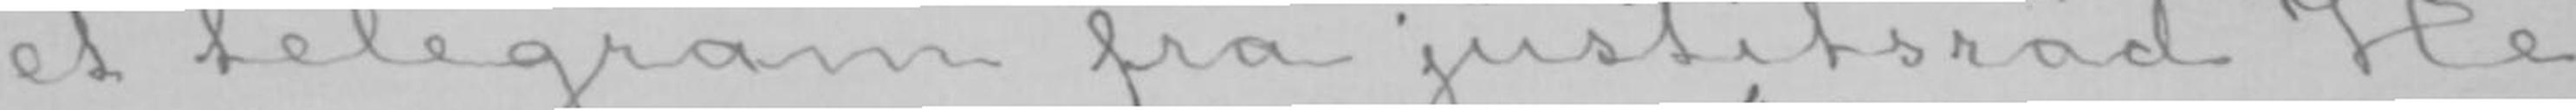et telegram fra justitsråd He-
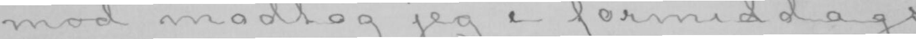mod modtog jeg i formiddags
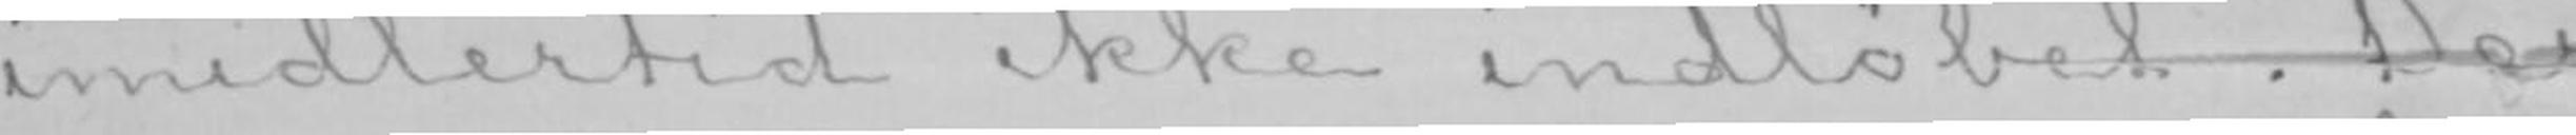imidlertid ikke indløbet. Deri-
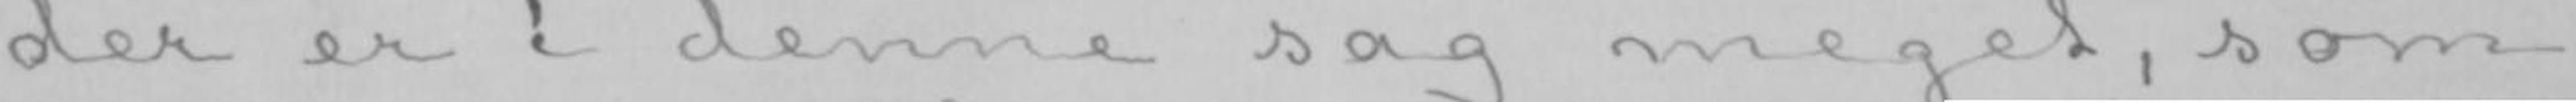der er i denne sag meget, som
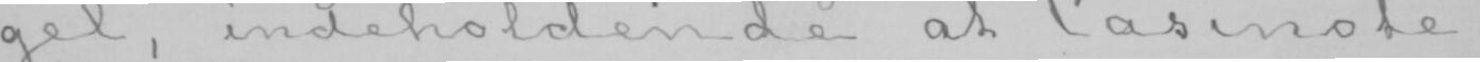gel, indeholdende at «Casinote-
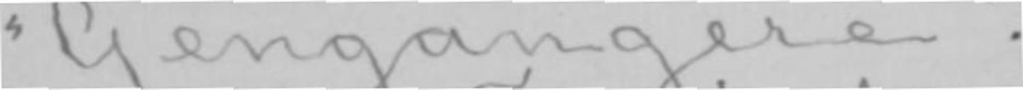«Gengangere».
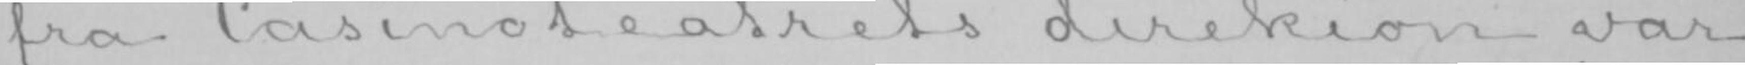fra Casinoteatrets direkion var
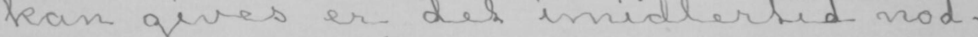kan gives er det imidlertid nød-
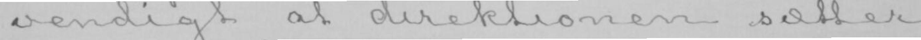vendigt at direktionen sætter
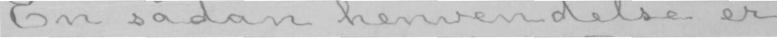En sådan henvendelse er
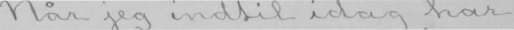Når jeg indtil idag har
